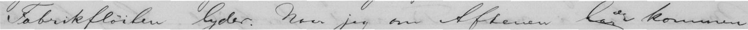Fabrikfløiten lyder. Naar jeg om Aftene har er kommen
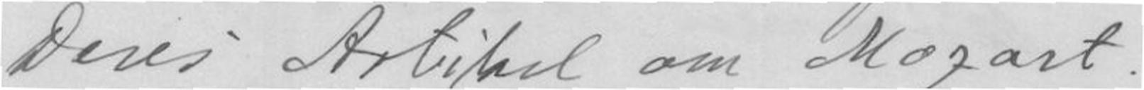Deres Artikel om Mozart.
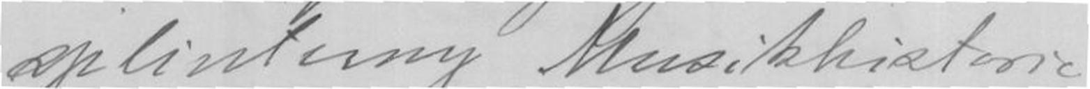splinterny Musikhistorie
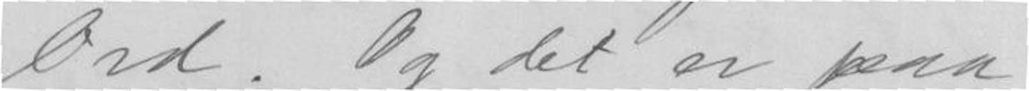Ord. Og det er paa
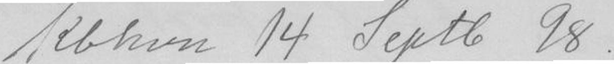København 14 Septb. 98.
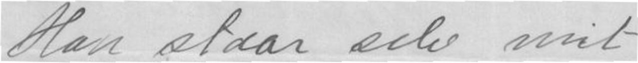Han staar selv mit
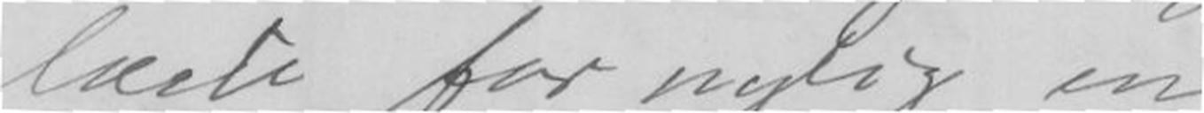læste for nylig en
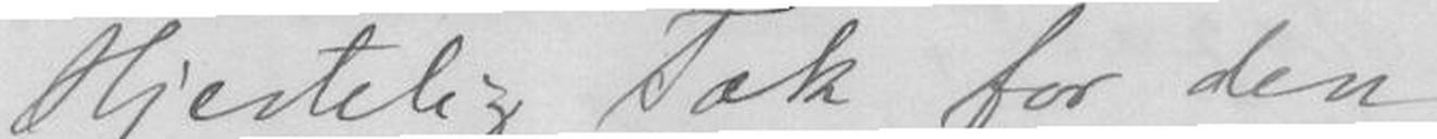Hjertelig Tak for den
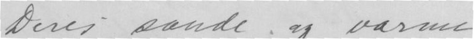Deres sande og varme
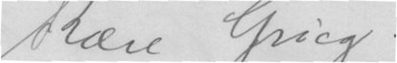Kære Grieg!
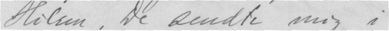Hilsen, De sendte mig i
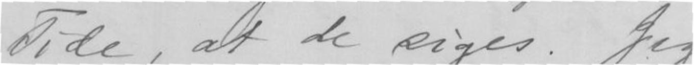Tide, at de siges. Jeg
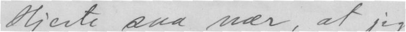Hjerte saa nær, at jeg
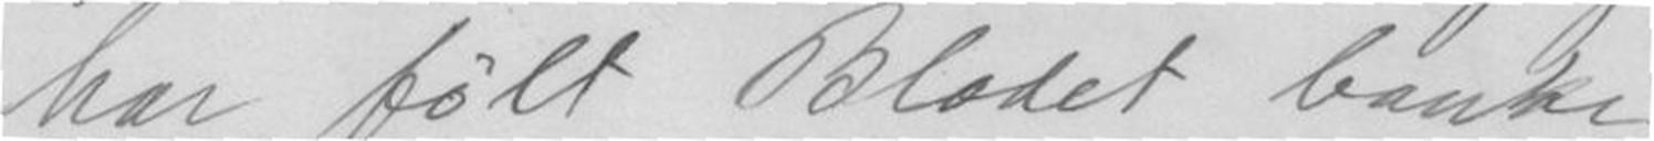har følt Blodet banke
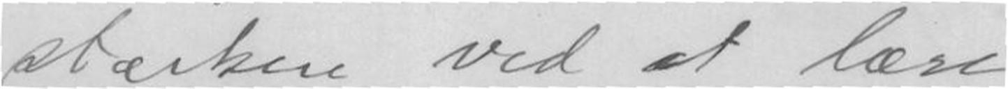stærkere ved at lære
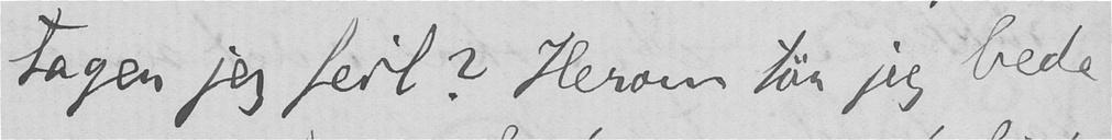tager jeg feil? Herom tør jeg bede
